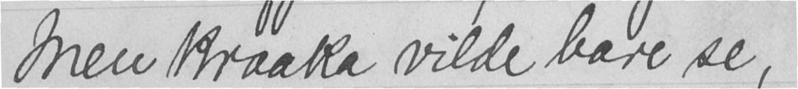Men kraaka vilde bare se,
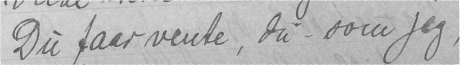Du faar vente, du - som jeg,
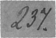237.
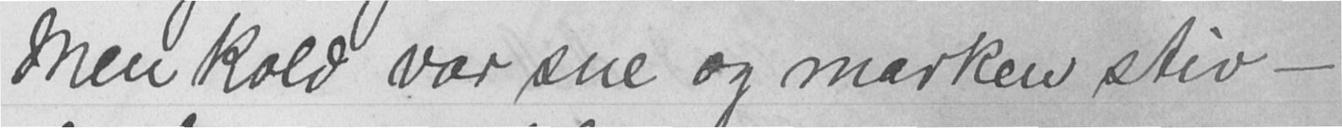Men kold var sne og marken stiv -
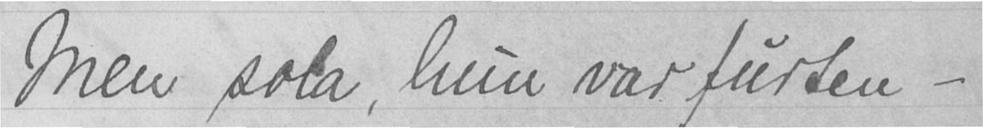Men sola, hun var furten -
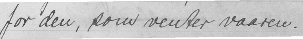for den, som venter vaaren.
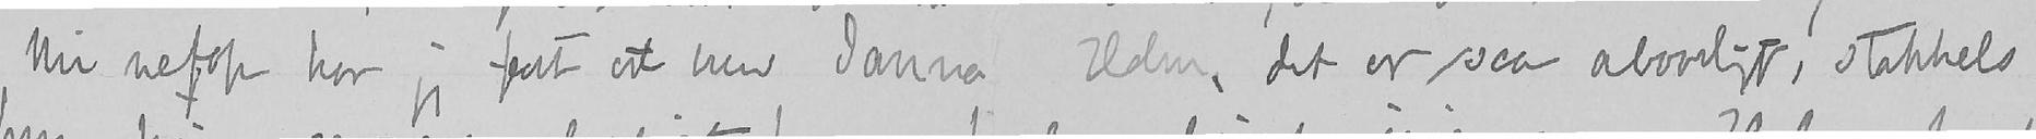Nu netop har jeg faat et brev Janna Holm, det er saa alvorligt, stakkels
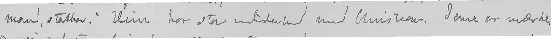mand, stakkar.» Hun har stor medlidenhed med Christian. Jane er mærke-
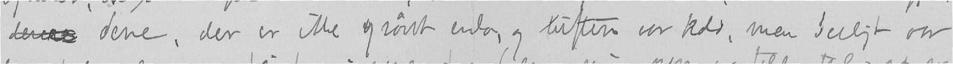deres dene, der er ikke grønt enda, luften var kold, men deiligt var
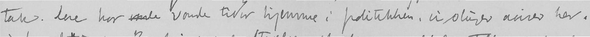tak. Dere har vonle vonde tider hjemme i politikken, vi sluge aviser her,
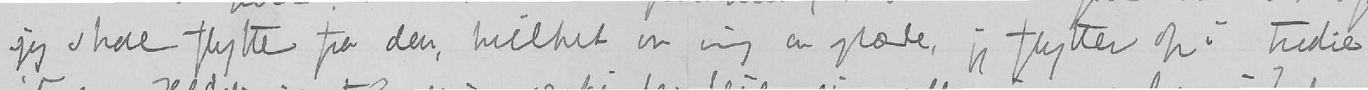jeg skal flytte fra den, hvilket er mig en glæde, jeg flytter op i tredie
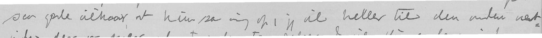saa gode vilkaar, at hun sa mig op, jeg vil heller til den anden vært-
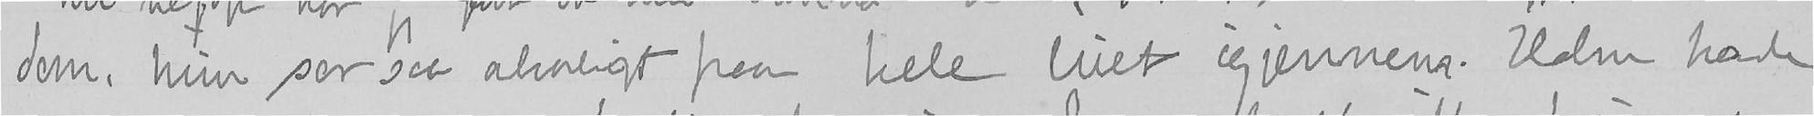dem, hun ser saa alvorligt paa hele livet igjennem. Holm havde
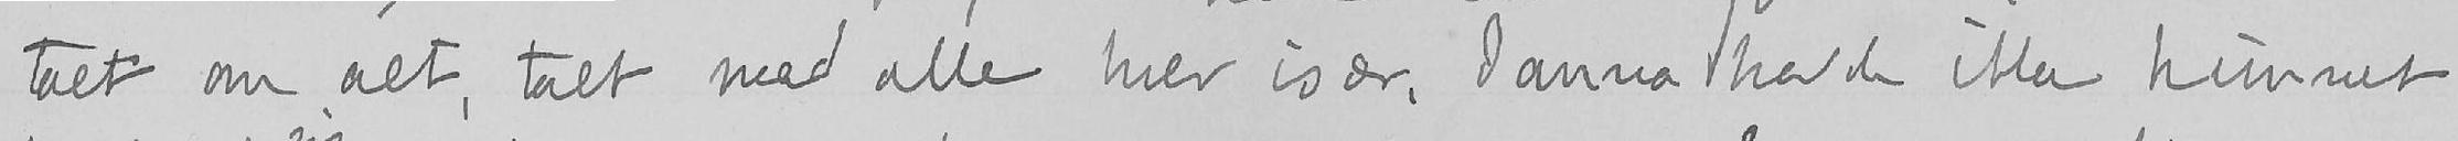talt om alt, talt med alle hver især, Janna Holm havde ikke kunnet
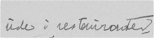ude i restauranter,
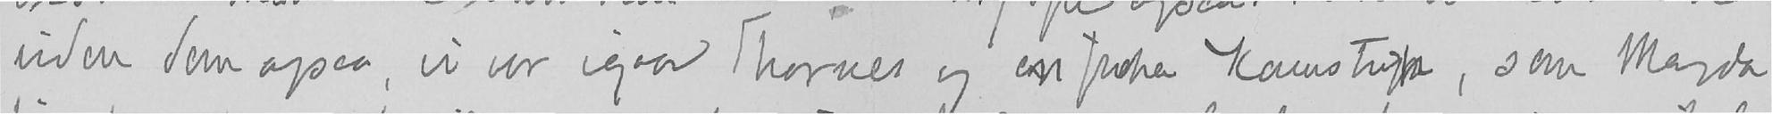uden dem ogsaa, vi var igaar Thornes og en frøken Kamstrup, som Magda
